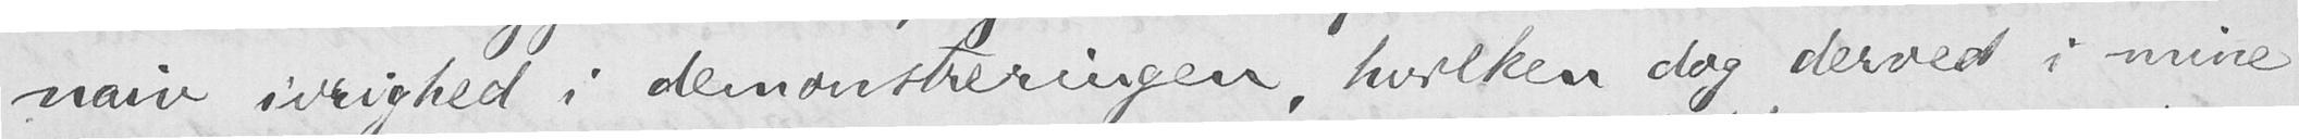naiv ivrighed i demonstreringen, hvilken dog derved i mine
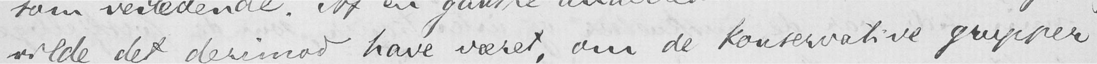vilde det derimod have været, om de konservative grupper
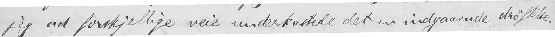jeg ad forskjellige veie underkastede det en indgaaende drøftelse.
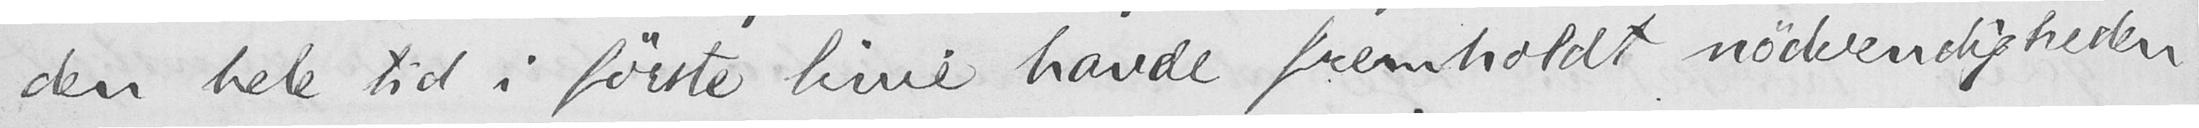den hele tid i første linie havde fremholdt nødvendigheden
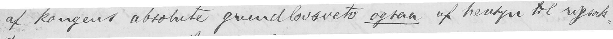af kongens absolute grundlovsveto ogsaa af hensyn til rigsak-
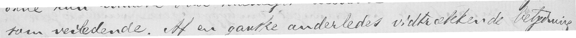som veiledende. Af en ganske anderledes vidtrækkende betydning
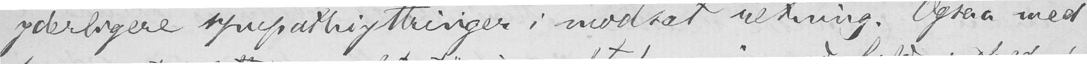yderligere sympathiyttringer i modsat retning. Ogsaa med
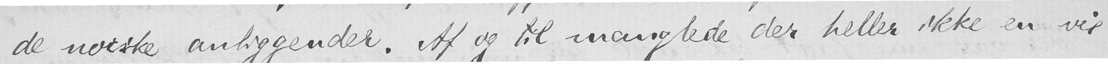de norske anliggender. Af og til manglede der heller ikke en vis
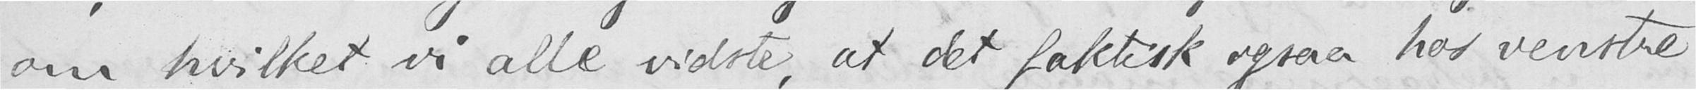om hvilket vi alle vidste, at det faktisk ogsaa hos venstre
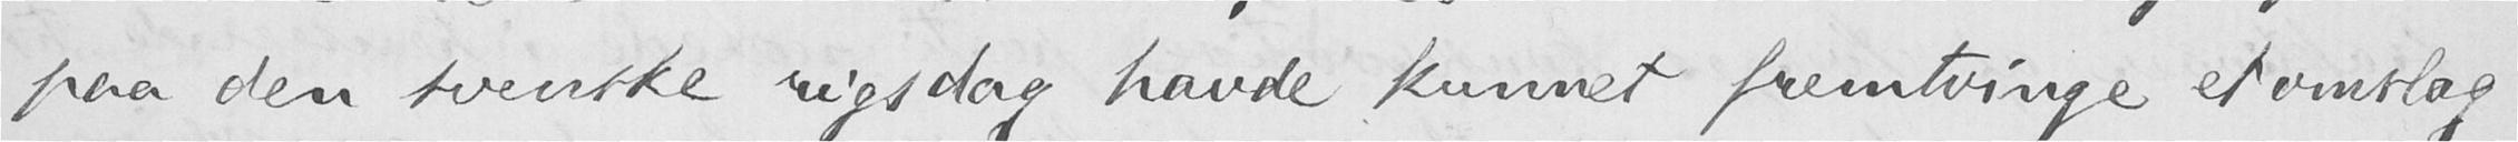paa den svenske rigsdag havde kunnet fremtvinge et omslag
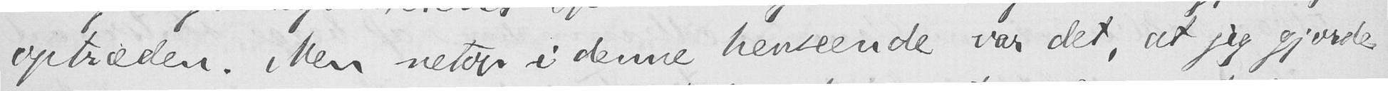optræden. Men netop i denne henseende var det, at jeg gjorde
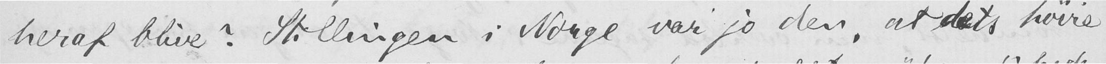heraf blive? Stillingen i Norge var jo den, at dets høire
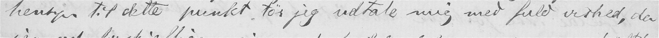hensyn til dette punkt, tør jeg udtale mig med fuld vished, da
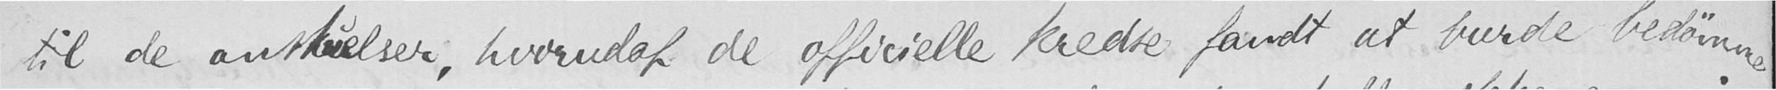til de anskuelser, hvorudaf de officielle kredse fandt at burde bedømme
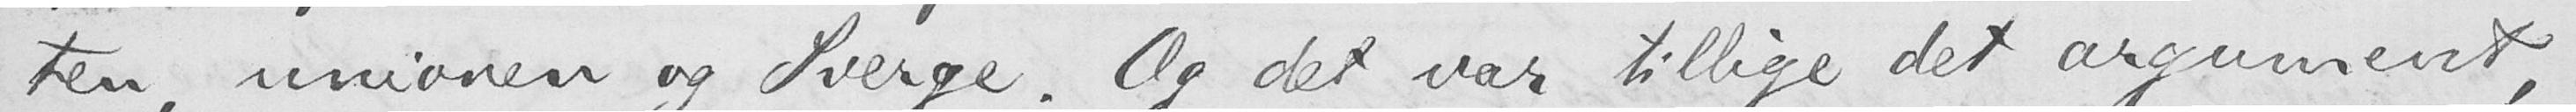ten, unionen og Sverge. Og det var tillige det argument,
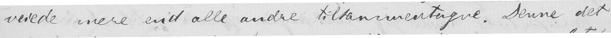veiede mere end alle andre tilsammentagne. Denne det
